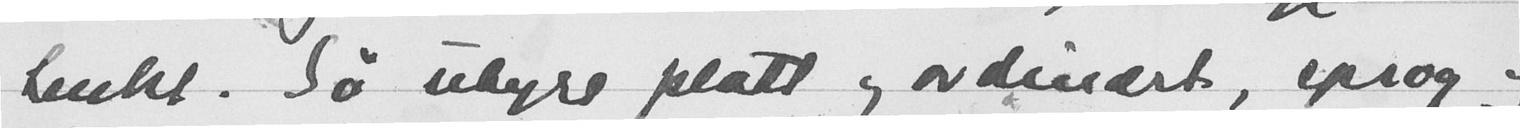tenkt. Så uhyre platt og ordinært, sprog og
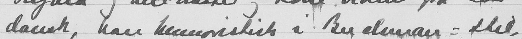dansk, han humoristisk i Berchman - stil
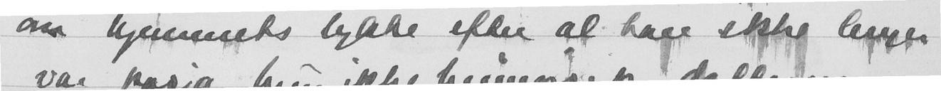om hjemmets lykke efter at han ikke lenger
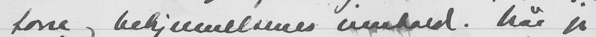tone og bekjennelsenes innfald. Når jeg
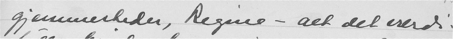gjemmesteder, Regine - alt det verdi-
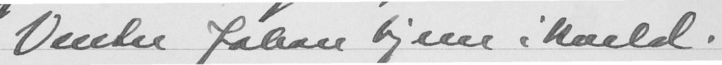Venter Johan hjem i kveld.
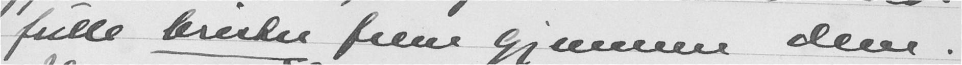fulle brister frem gjennom Dem.
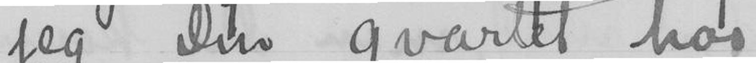jeg Din qvartet hos
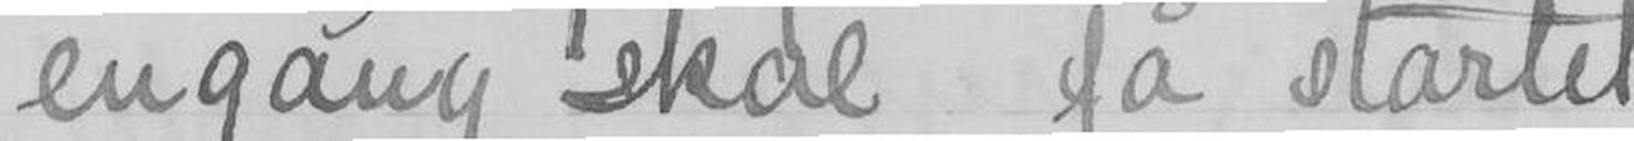engang skal få startet
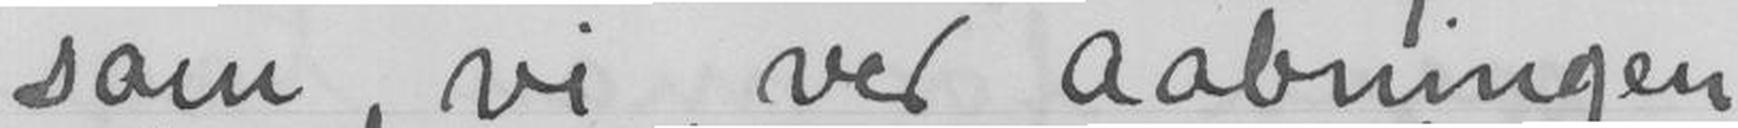som vi ved aabningen
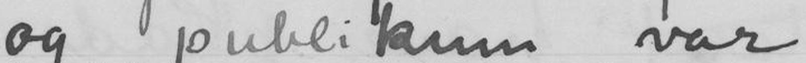og publikum var
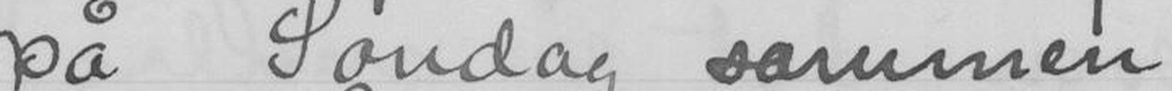på Søndag sammen
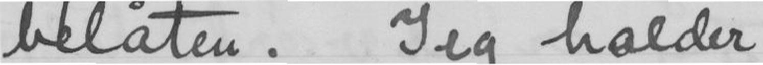belåten. Jeg holder
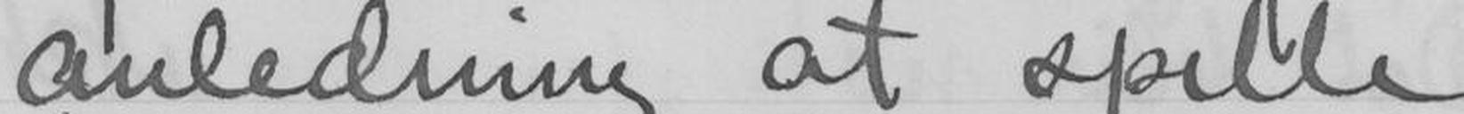anledning at spille
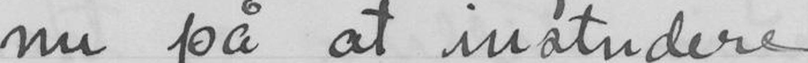nu på at instudere
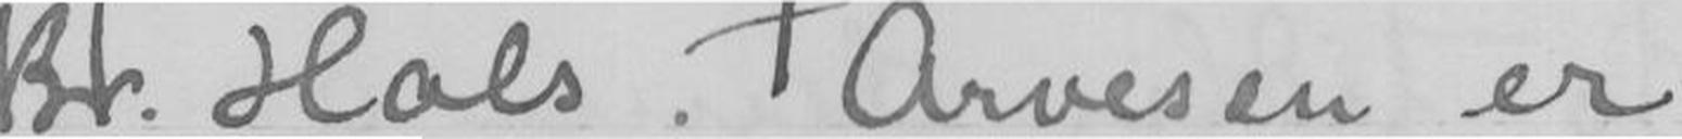Br. Hals. Arvesen er
